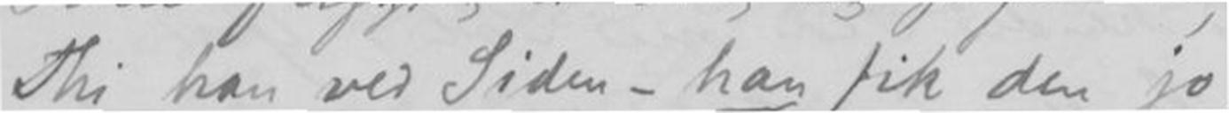Thi han ved Siden - han fik den jo,
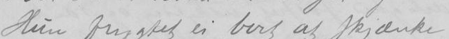Hun frygtet ei bort at skjænke,
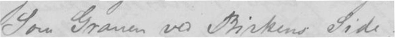Som Granen ved Birkens Side;
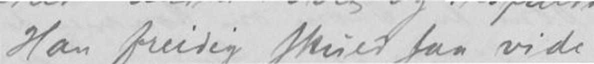Han freidig skued saa vide
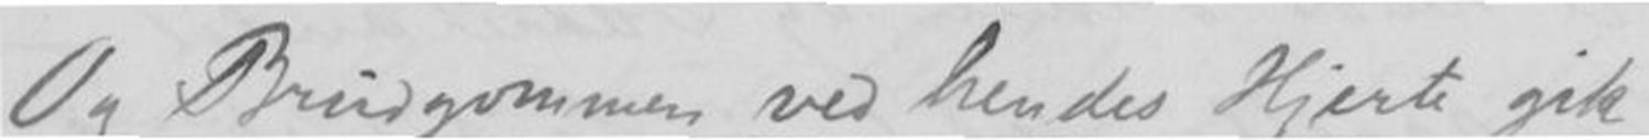Og Brudgommen ved hendes Hjerte gik
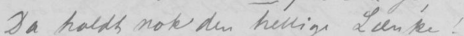Da holdt nok den hellige Lænke !
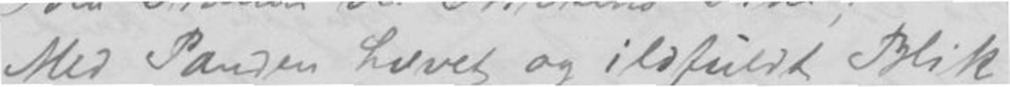Med Panden hevet og ildfuldt Blik
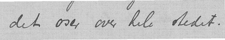det oser over hele stedet.
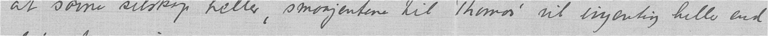at savne selskap heller, smaajentene til Thomas' vil ingenting heller end
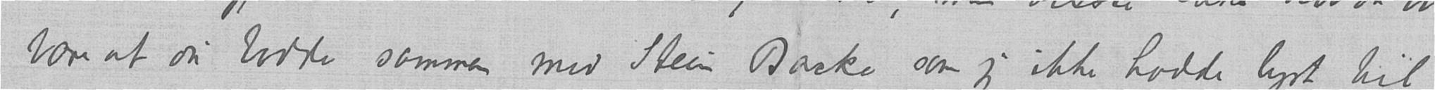bare at du bodde sammen med Stein Backe som jeg ikke hadde lyst til
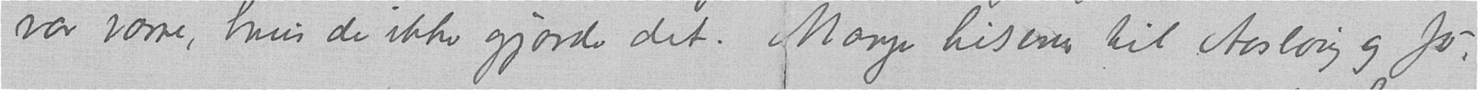var værre, hvis de ikke gjorde det. Mange hilsener til Aaslaug og Jo.
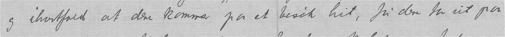og ihvertfald at dere kommer paa et besøk hit, før dere tar ut paa
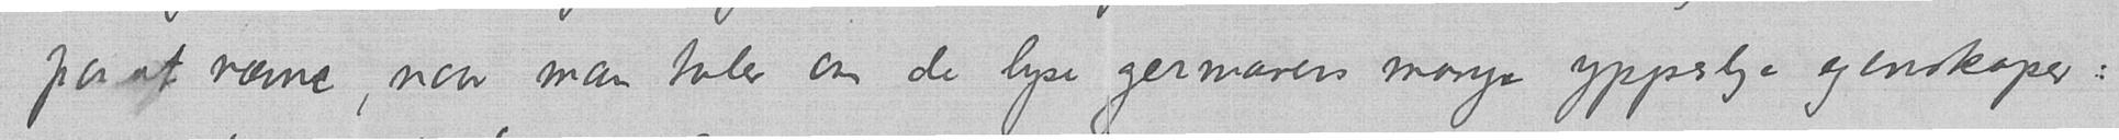paa at nævne, naar man taler om de lyse germaners mange ypperlige egenskaper:
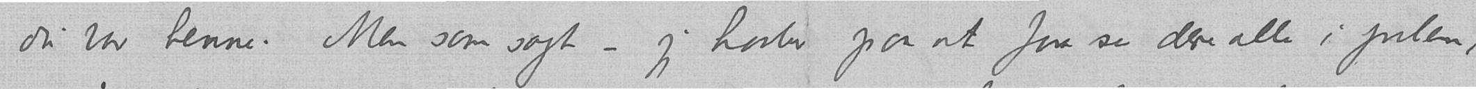du var henne. Men som sagt – jeg haaber paa at faa se dere alle i julen,
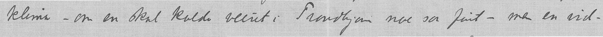klima – om en skal kalde veiret i Trondhjem noe saa fint – men en vid-
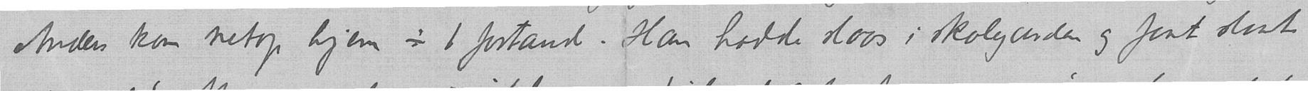Anders kom netop hjem  ÷ 1 fortand. Han hadde slaas i skolegaarden og faat slaat
